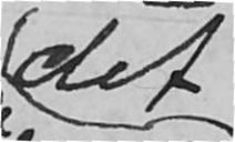det
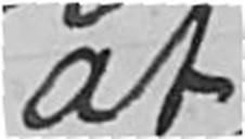at
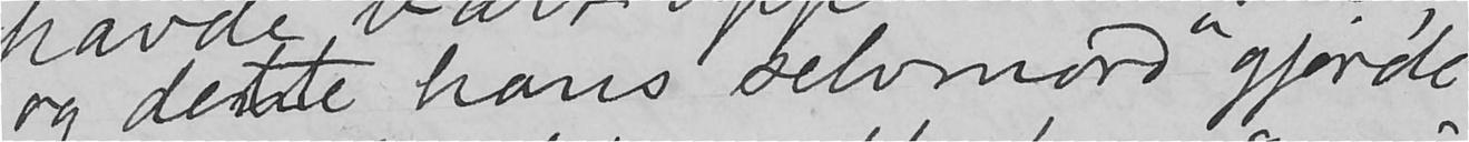og dette hans selvmord gjorde
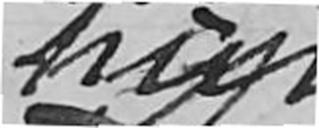hun
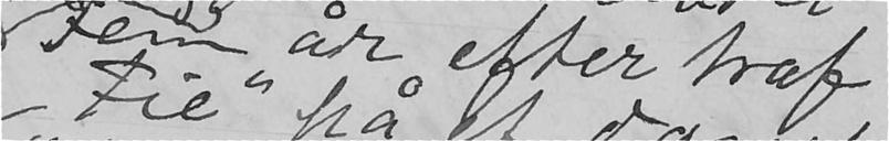Fem år efter traf
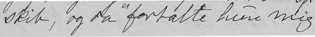skib, og da fortalte hun mig
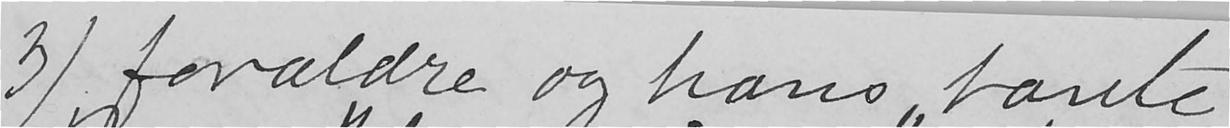3) forældre og hans "tante
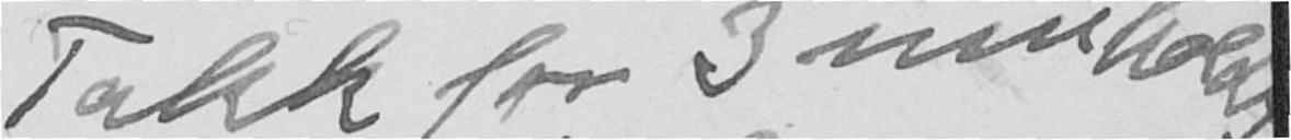Takk for 3 innholds-
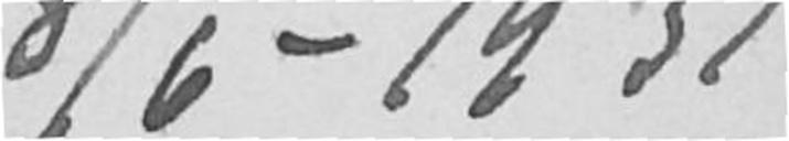8/6-1937
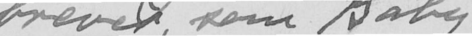brever, som Baby
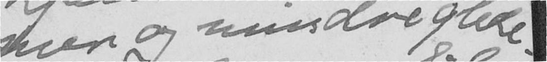skrep og minsdre glede-
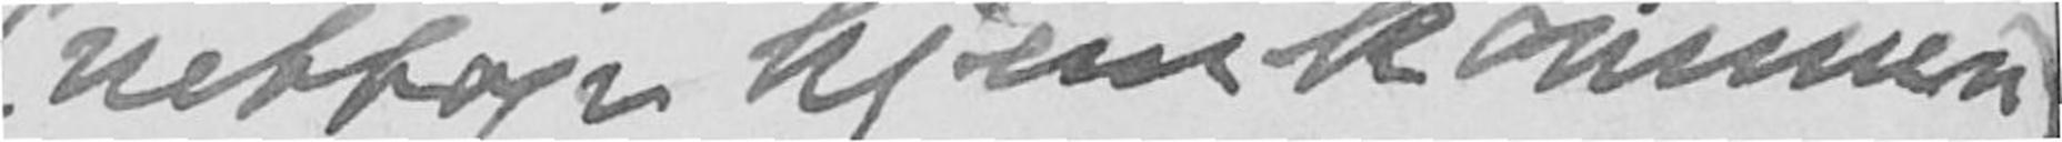(nettop hjemkommen)
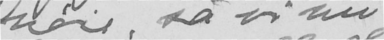nøie, så vi nu
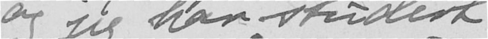og jeg har studert
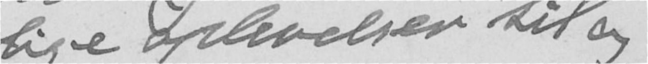lige oplevelser til og
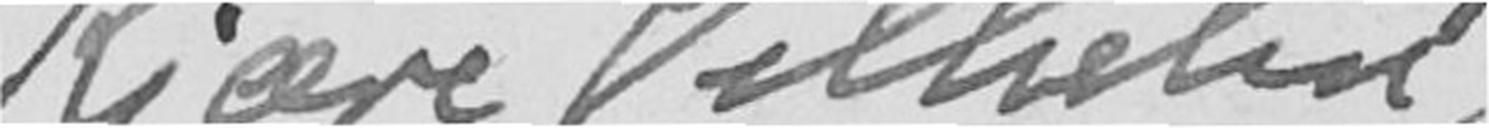Kjære Vilhelm,
